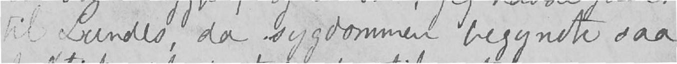til Lundes, da sygdommen begyndte saa
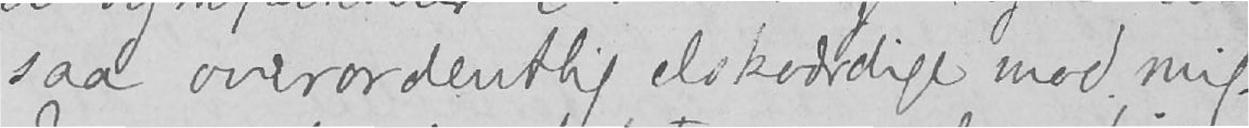saa overordentlig elskværdige mod mig.
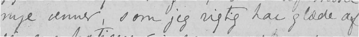nye venner, som jeg rigtig har glæde af,
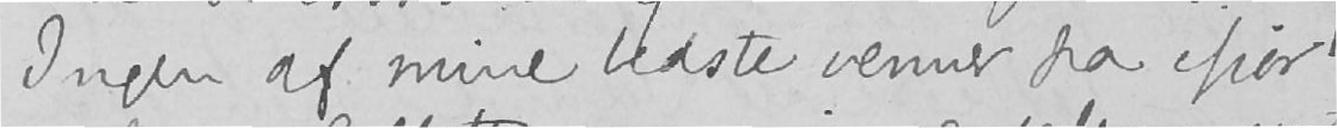Ingen af mine bedste venner fra ifjor
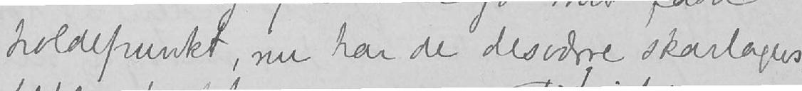holdepunkt, nu har de desværre skarlagens-
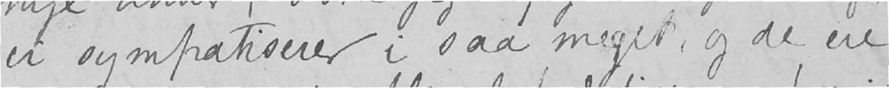vi sympatiserer i saa meget, og de ere
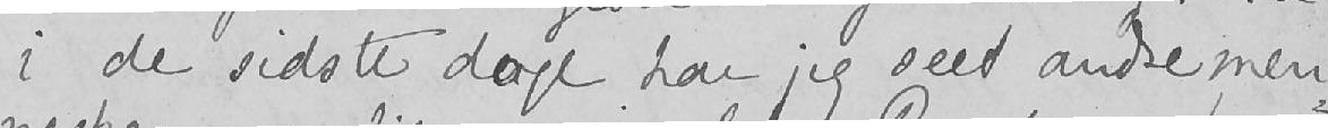i de sidste dage har jeg seet andre men-
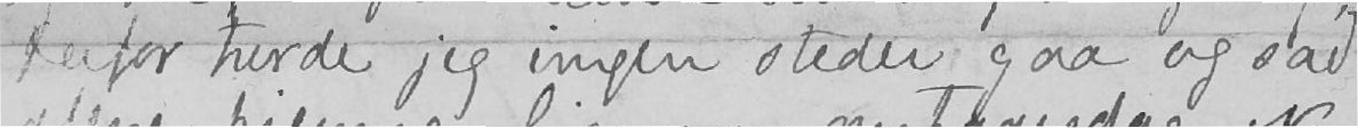derfor turde jeg ingen steder gaa og sov
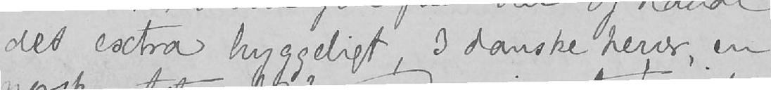det extra hyggeligt, 3 danske herrer, en
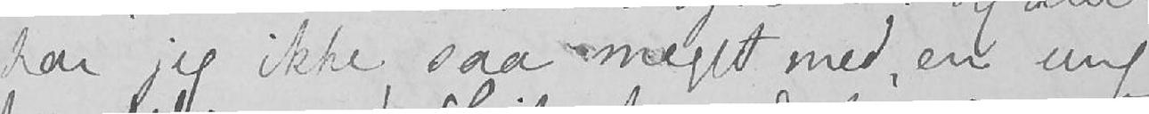har jeg ikke saa meget med, en ung
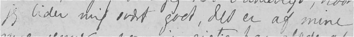jeg lider mig svært godt, det er af mine
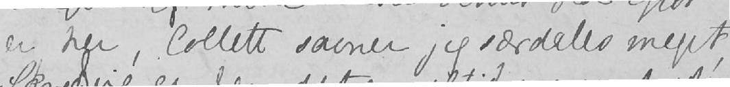er her, Collett savner jeg særdeles meget,
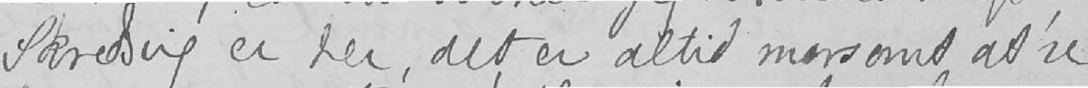Skredsvig er her, det er altid morsomt at se
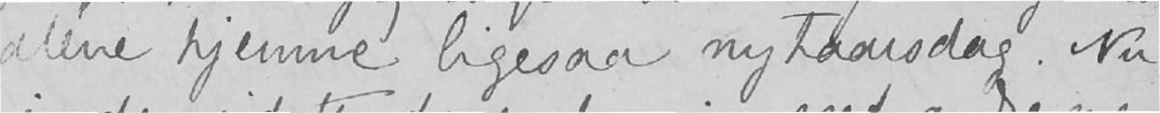alene hjemme ligesaa nytaarsdag. Nu
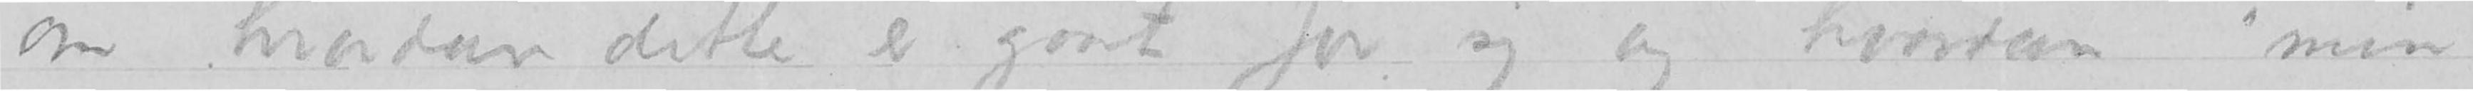om hvordan dette er gaat for sig og hvordan «min
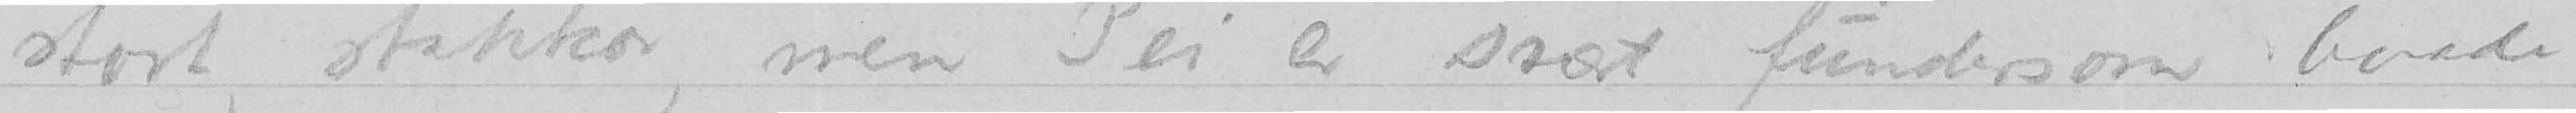stort, stakkar, men Pei er svært fundersom baade
